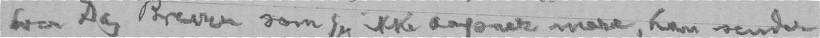hver Dag Brever som jeg ikke aapner mere, han sender
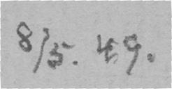8/5. 49.
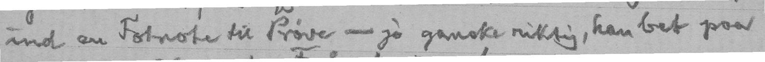ind en Fotnote til Prøve - jo ganske riktig, han bet paa
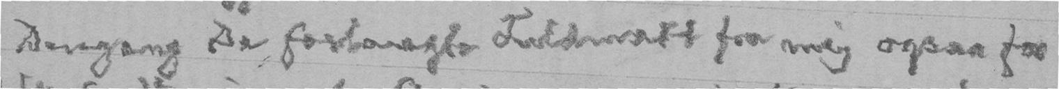Dengang De forlangte Fuldmakt fra mig ogsaa for
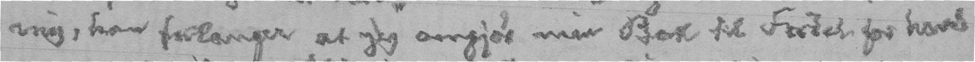mig, han forlanger at jeg omgjør min Bok til Fordel for hans
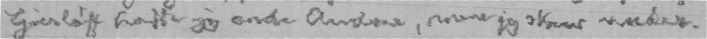Gierløff hadde jeg onde Anelser, men jeg skrev under.
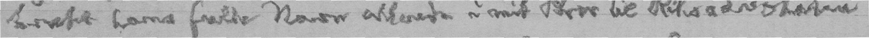brukt hans fulde Navn allerede i mit Brev til Riksadvokaten
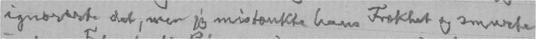ignorerte det, men jeg mistænkte hans Frækhet og smurte
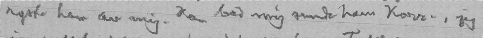ryste ham av mig. Han bad mig sende ham Korr., jeg
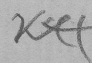KH
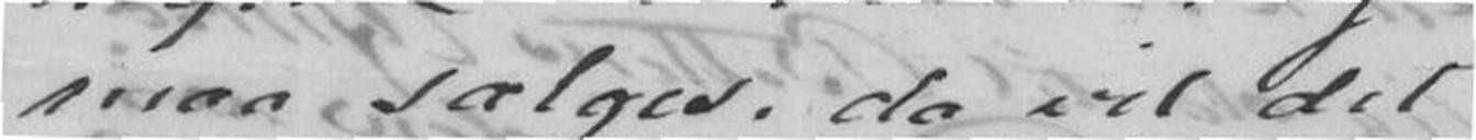maa sælges, da vil det
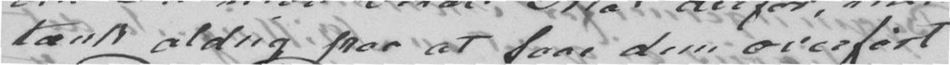tænk aldrig paa at faae dem overført
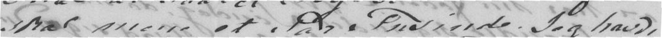skal mene et Par Tusinde. Jeg havde
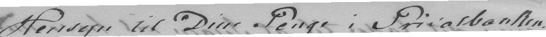Hensyn til Dine Penge i Privatbanken.
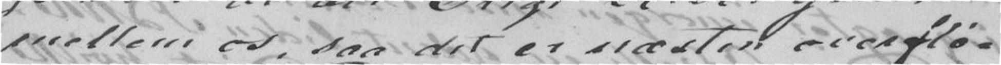mellem os, saa det er næsten overflø-
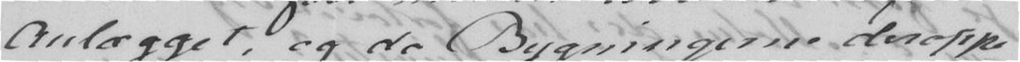Anlægget, og da Bygningerne deroppe
